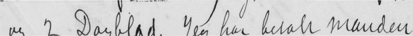og 2 Dagblad. Jeg har betalt Manden
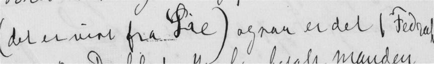(det er mine fra Lie) ogsaa er det 1 Fædrelandsvennen
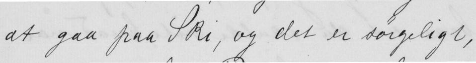at gaa paa Ski, og det er sørgeligt,
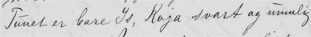Tunet er bare Is, Koja svart og umulig
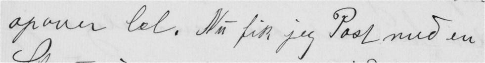opover lel. Nu fik jeg Post med en
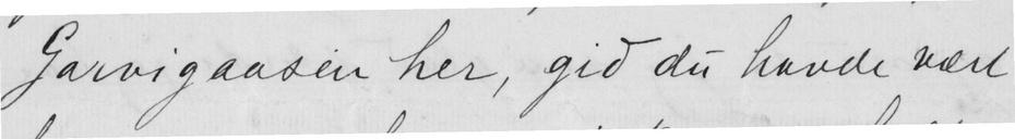Garvigaarden her, gid du havde vært
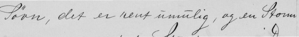Søvn det er rent umulig, og en Storm
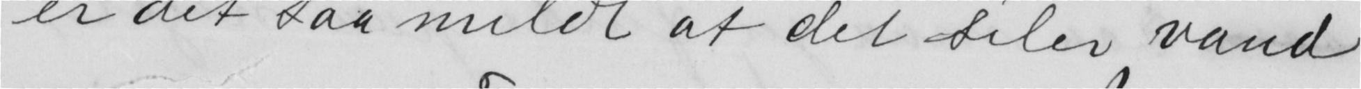er det saa mildt at det siler vand
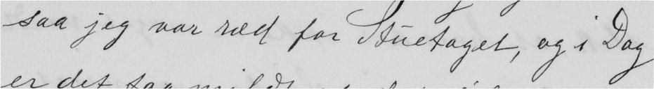saa jeg var ræd for Stuetaget, og i Dag
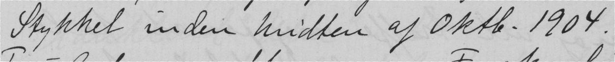Stykket inden midten af Oktb. 1904.
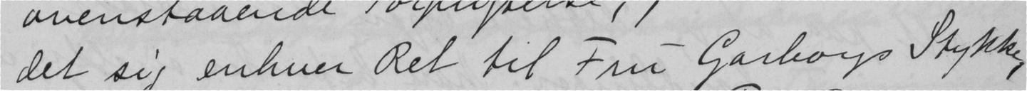det sig enhver Ret til Fru Garbørgs Stykke,
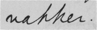vakker.
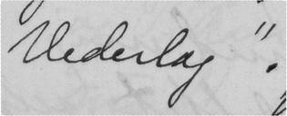Vederlag".
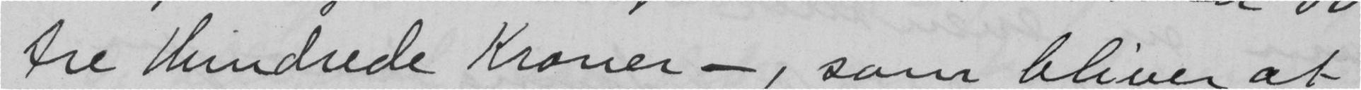tre Hundrede Kroner -, som bliver at
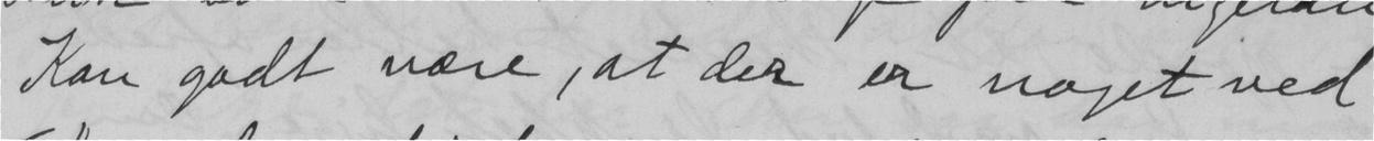Kan godt være, at der er noget ved
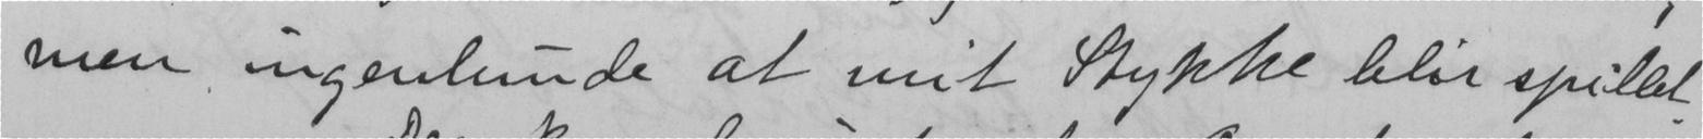men ingenlunde at mit Stykke blir spillet.
